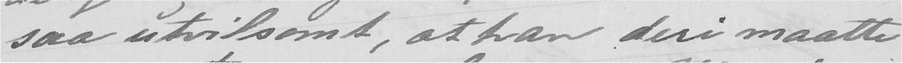saa utvilsomt, at han deri maatte
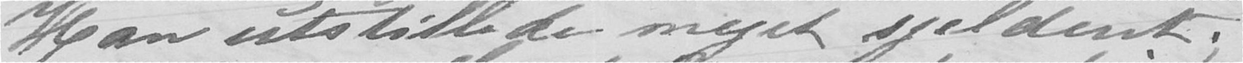Han utstillede meget sjeldent,
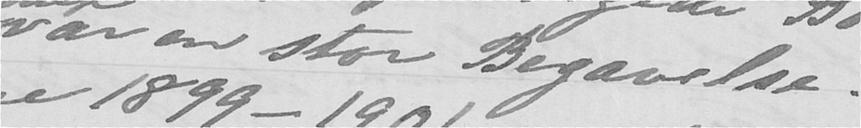var en stor Begavelse.
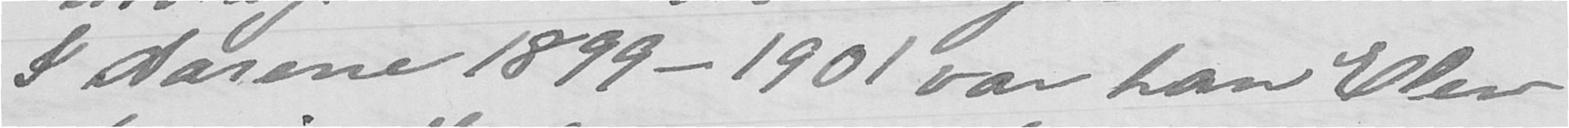I Aarene 1899-1901 var han Elev
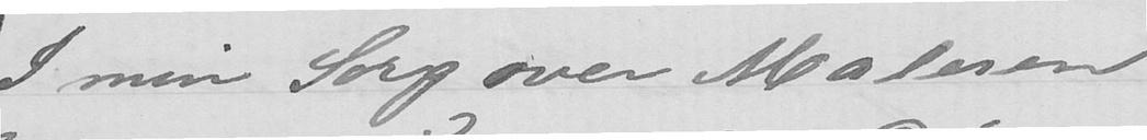I min Sorg over Maleren
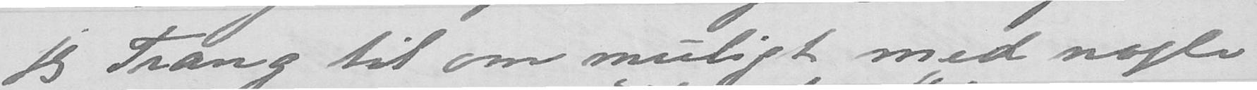jeg Trang til om muligt med nogle
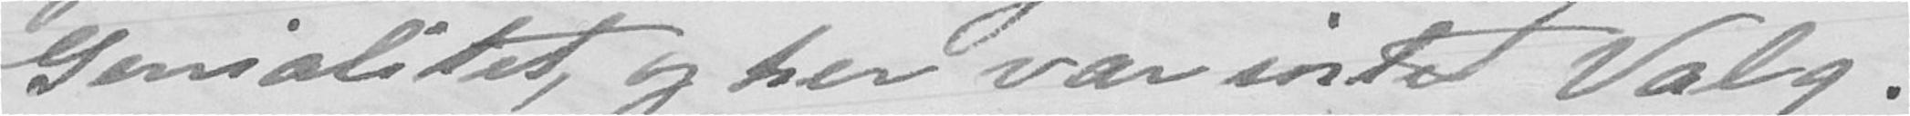Genialitet, og her var intet Valg.
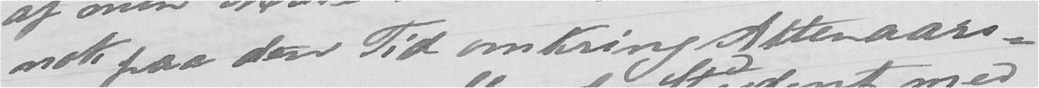nok paa den Tid omkring Attenaars-
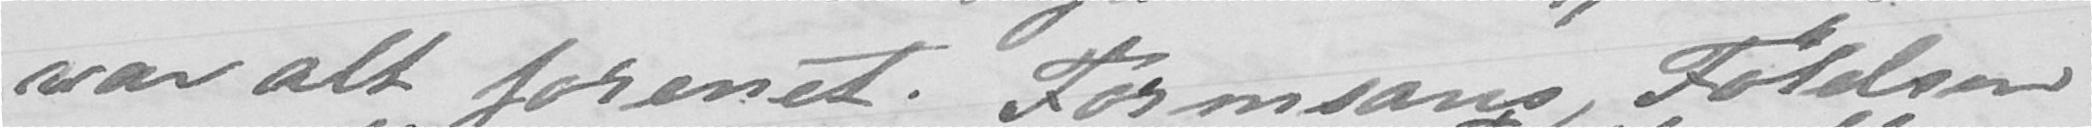var alt forenet. Formsans, Følelsen
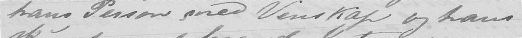hans Person med Venskap og hans
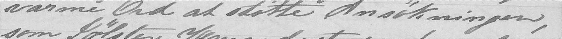varme Ord at støtte Ansøkningen,
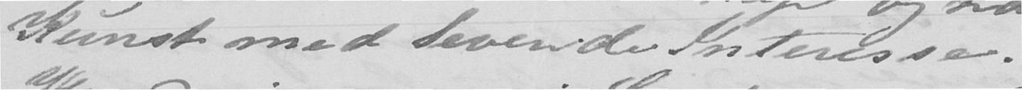Kunst med levende Interesse.
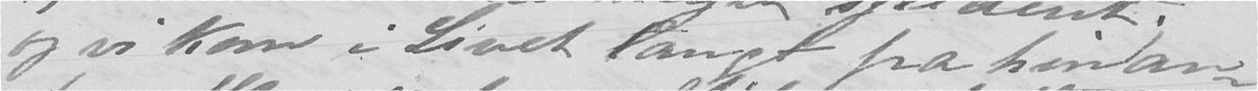og vi kom i Livet langt fra hinan-
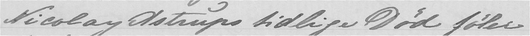Nicolay Astrups tidlige Død føler
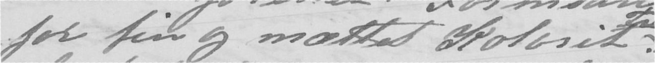for fin og mættet Kolorit.
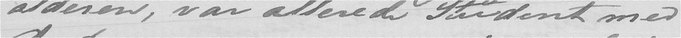alderen, var allerede Student med
